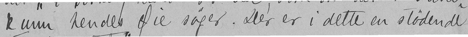kum hendes Øie søger. Der er i dette en stødende
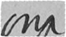om
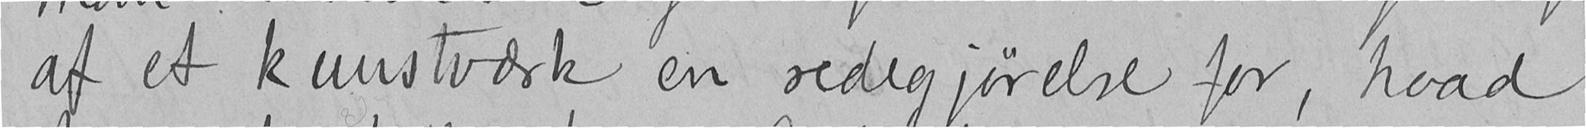af et kunstværk en redegjørelse for, hvad
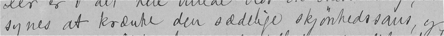synes at krænke den sædelige skjønhedssans, og
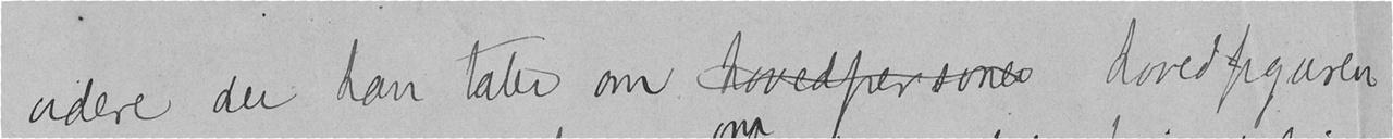videre der han taler om hovedpersonen hovedfiguren
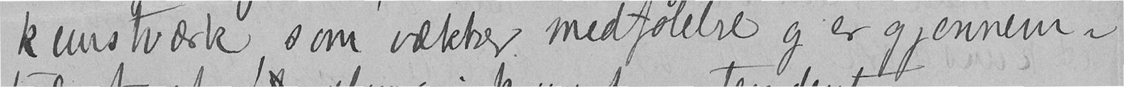kunstværk som vækker medfølelse og er gjennem-
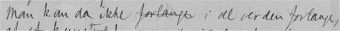Man kan da ikke forlange i al verden forlange,
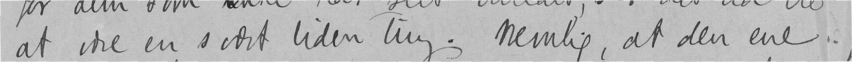at være en svært liden ting. Nemlig at den ene
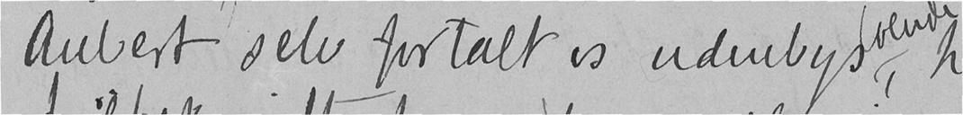Aubert selv fortalt os udenbysboende,
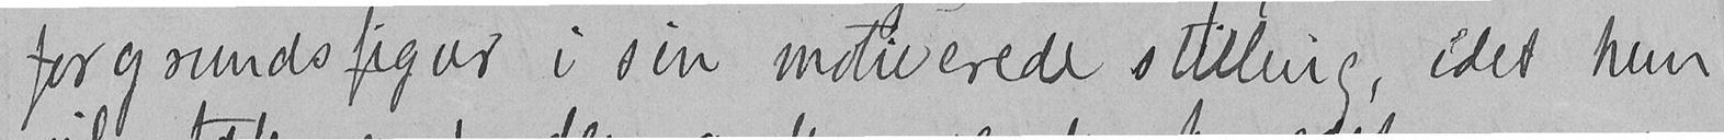forgrundsfigur i sin motiverede stilling, idet hun
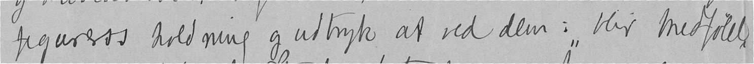figurers holdning og udtryk at ved dem: "blir medfølel-
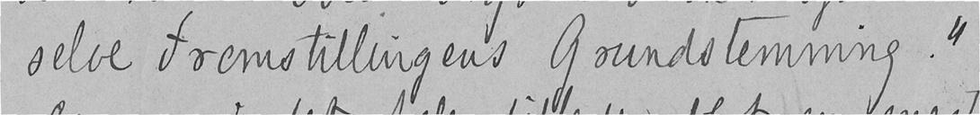selve Fremstillingens Grundstemning."
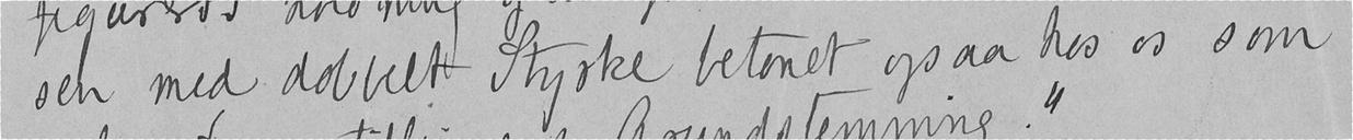sen med dobbelt Styrke betonet ogsaa hos os som
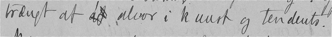trængt af af alvor i kunst og tendents.
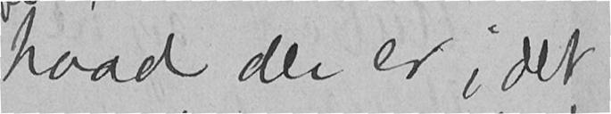hvad der er i det,
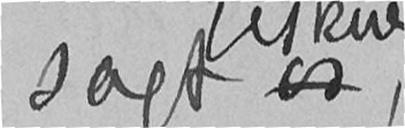sagt os,
